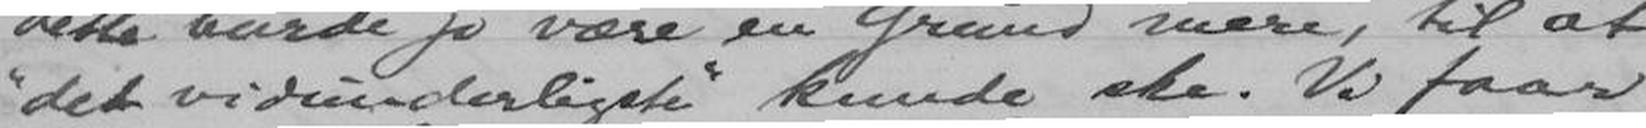"det vidunderligste" kunde ske. Vi faar
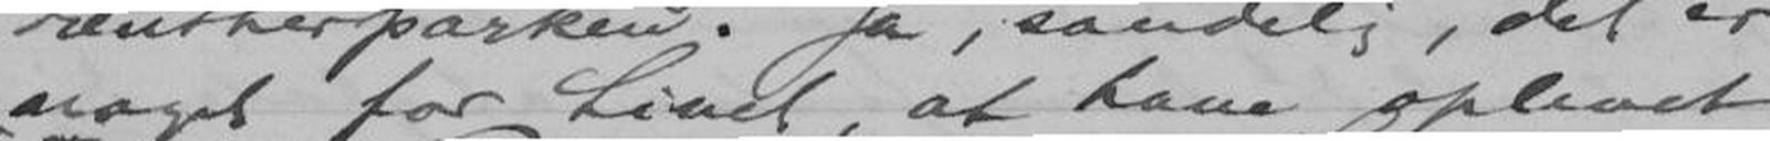noget for Livet, at have oplevet
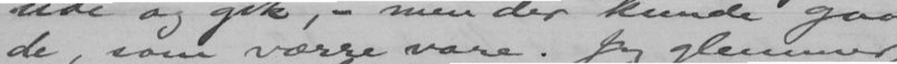de, som værre vare. Jeg glemmer
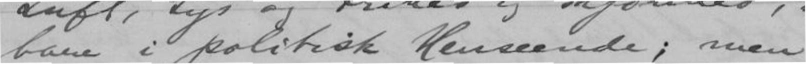bare i politisk Henseende; men
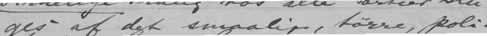ges af det smaalige, tørre, poli-
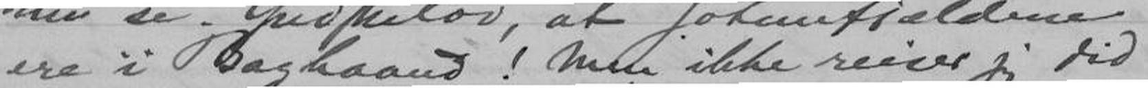ere i Baghaand! Men ikke reiser jeg did
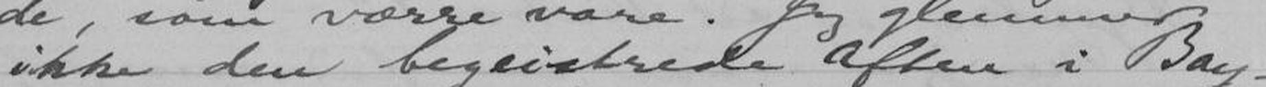ikke den begeistrede Aften i Bay-
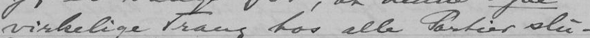virkelige Trang hos alle Parter slu-
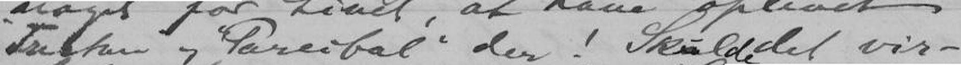"Tristan" og "Parcifal" der! Skulde det vir-
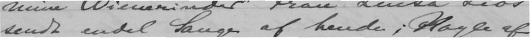sendt endel Sange af hende; Nogle af
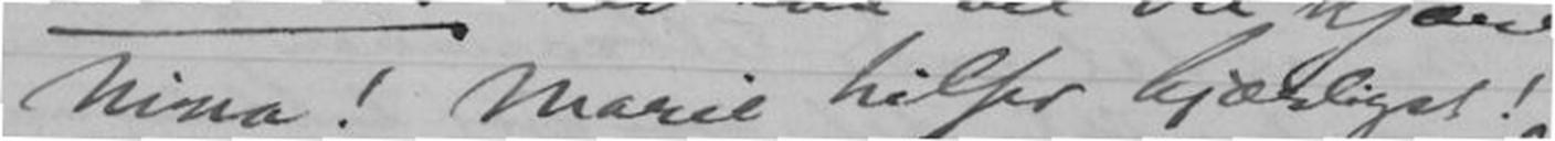Nina! Marie hilser kjærligst!
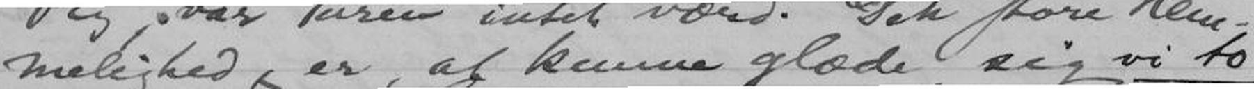melighed er, at kunne glæde sig vi to
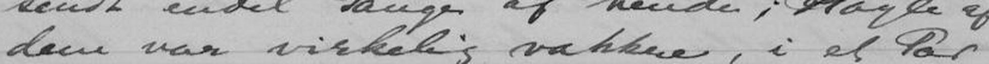af dem var det naturligvis Wagner
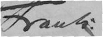Frants.
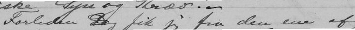Forleden Dag fik jeg fra den ene af
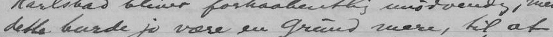dette burde jo være en Grund mere, til at
